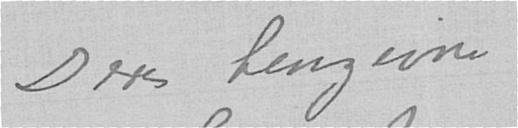Deres hengivne
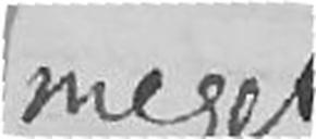meget
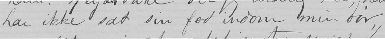har ikke sat sin fod indom min dør,
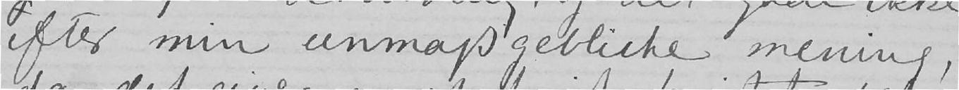efter min unmaßgebliche mening,
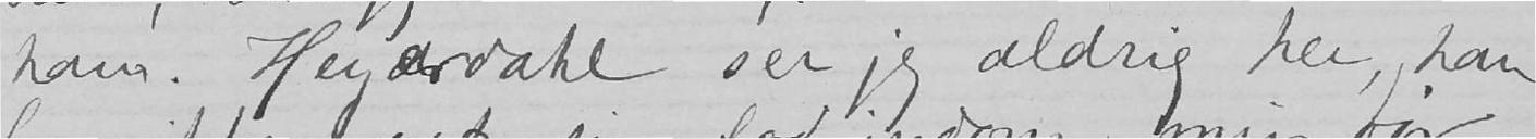ham. Heyerdahl ser jeg aldrig her, han
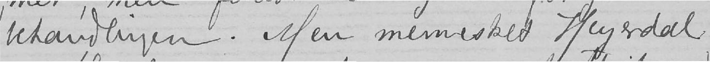behandlingen. Men mennesket Heyerdal
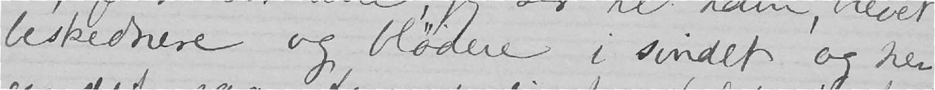beskednere og blødere i sindet og her
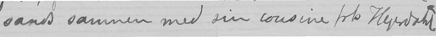sands sammen med sin cousine frk Heyerdahl.
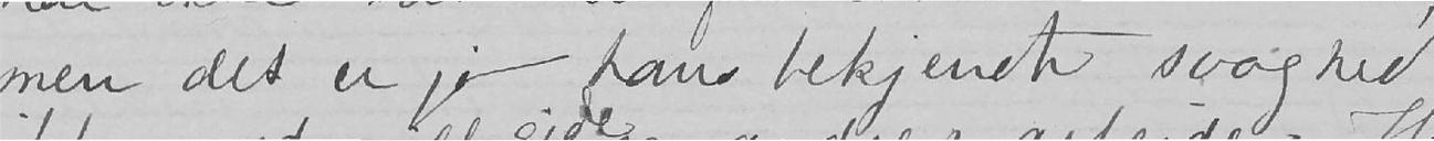men det er jo hans bekjendte svaghed
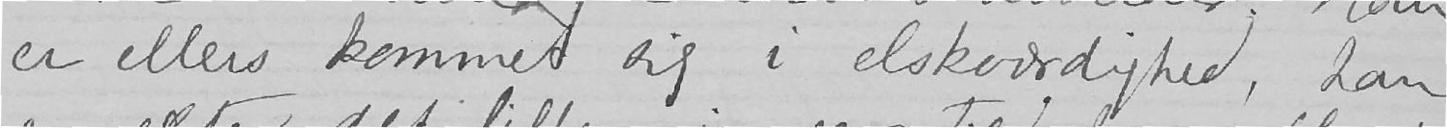er ellers kommet sig i elskværdighed, han
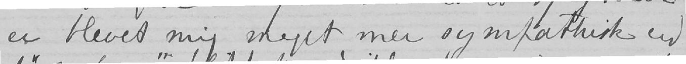er blevet mig meget mer sympathisk end
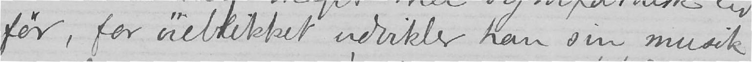før, for øieblikket udvikler han sin musik-
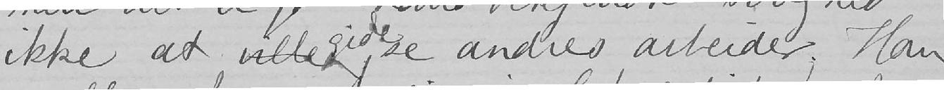ikke at ville gide se andres arbeider. Han
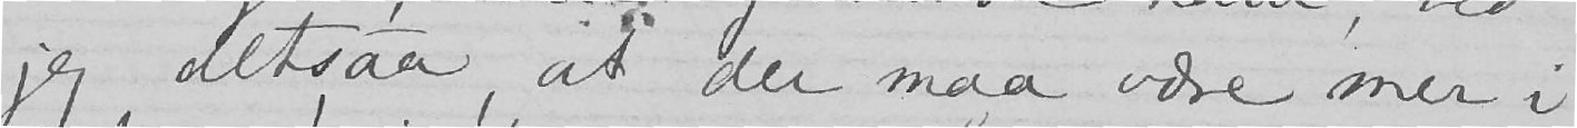jeg altsaa, at der maa være mer i
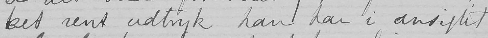ket rent udtryk han har i ansigtet.
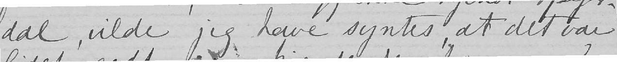dal, vilde jeg have syntes, at det var
